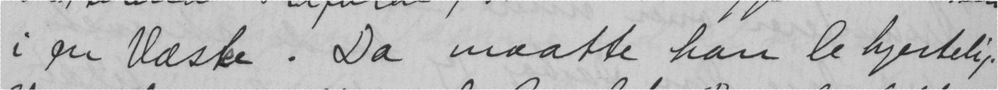i en Væske. Da maatte han le hjertelig.
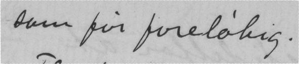som før foreløbig.
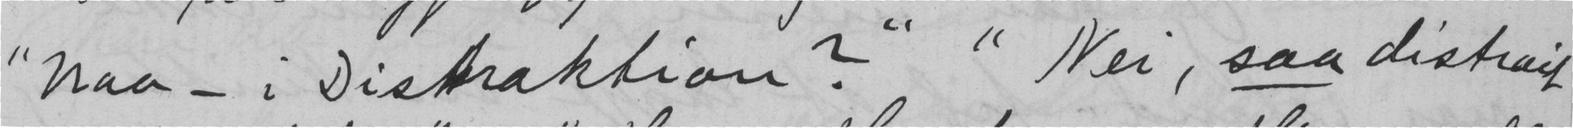"Naa - i Distraktion?" "Nei, saa distrait
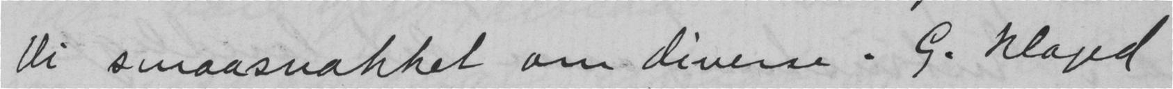Vi smaasnakket om diverse. G. klaged
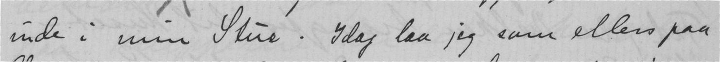inde i min Stue. Idag laa jeg som ellers paa
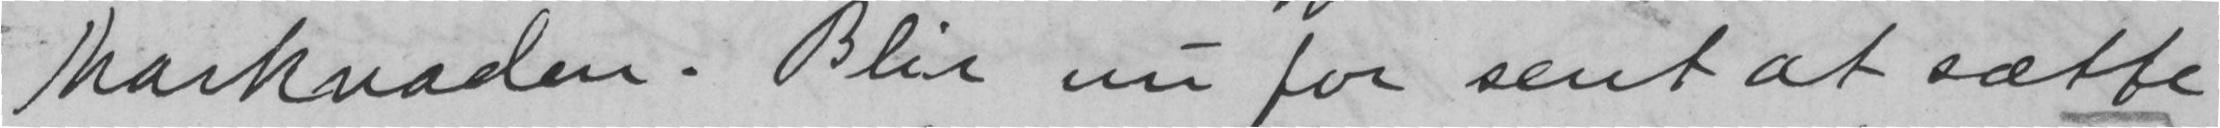Marknaden. Blir nu for sent at sætte
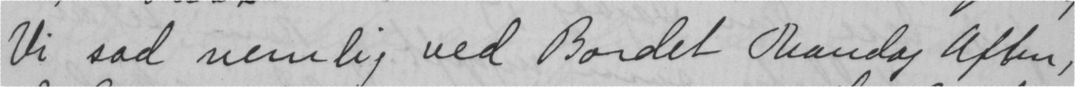Vi sad nemlig ved Bordet Mandag Aften,
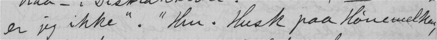er jeg ikke". "Hm. Husk paa Hønemelken,
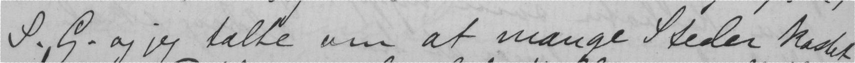S., G. og jeg talte om at mange Steder kastet
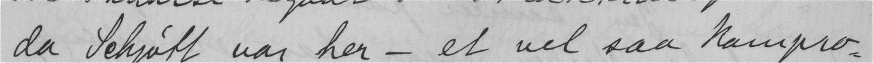da Schjøtt var her - et vel saa kompro-
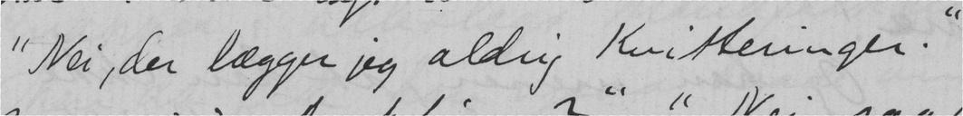"Nei, der lægger jeg aldrig Kvitteringer."
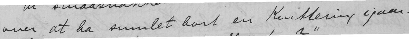over at ha sumlet bort en Kvittering igaar.
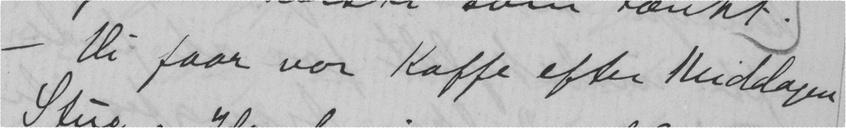- Vi faar vor Kaffe efter middagen
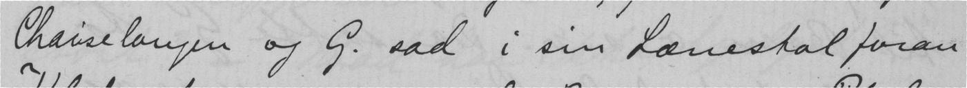Caiselongen og G. sad i sin Lænestol foran
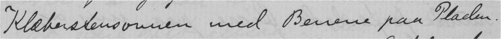Klæberstensovnen med Benene paa Pladen.
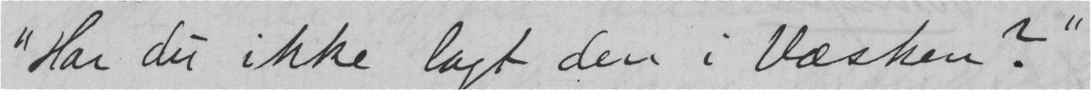"Har du ikke lagt den i væsken?"
